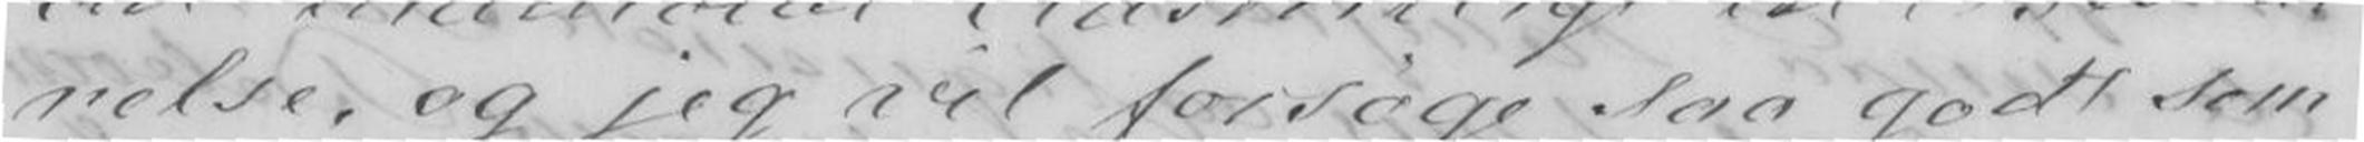relse, og jeg vil forsøge saa godt som
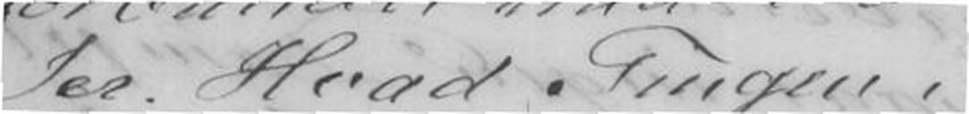Jer. Hvad Tingen
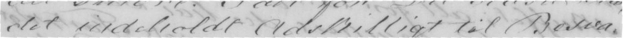det indeholdt Adskilligt til Besva-
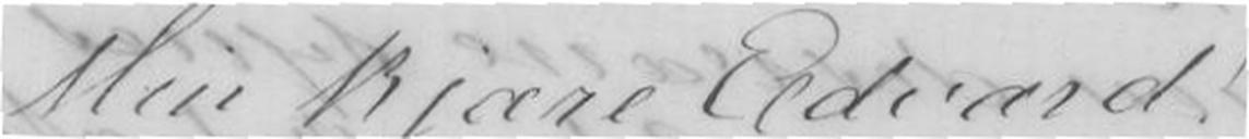Min kjære Edvard!
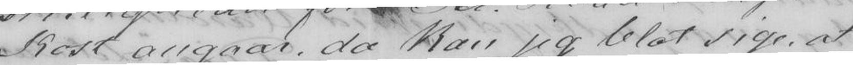Kost angaar, da kan jeg blot sige at
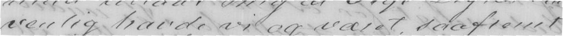venlig havde vi og været saafremt
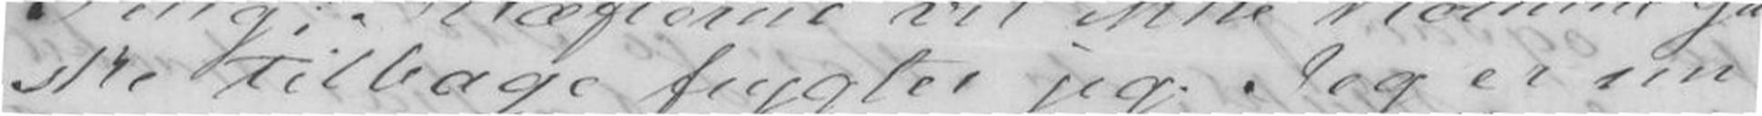ske tilbage frygter jeg. Jeg er nu
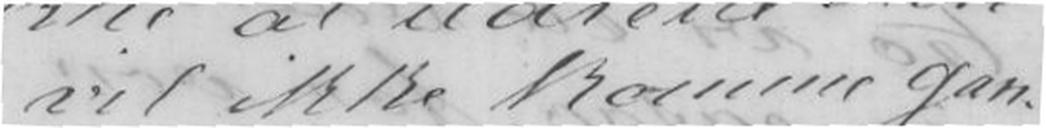vil ikke komme gan-
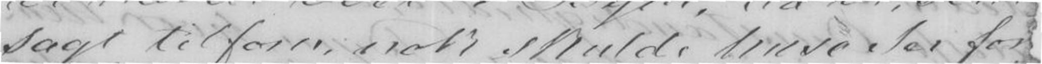sagt tilforn nok skulde huse Jer for
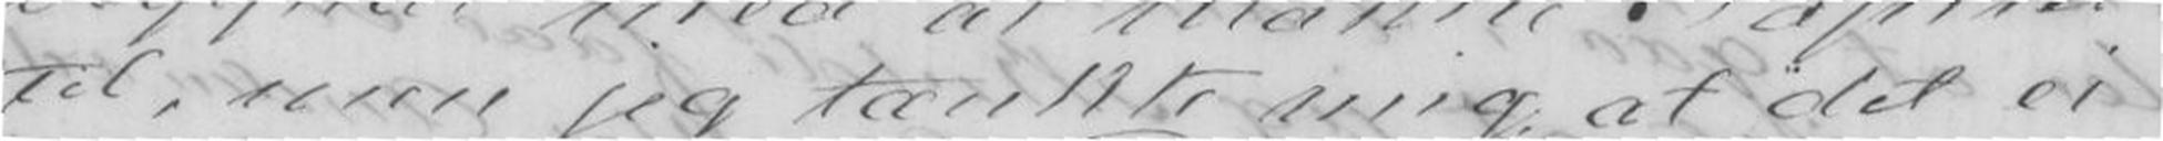til, men jeg tænkte mig at det ei
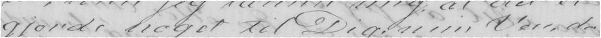gjorde noget til Dig, min Ven, da
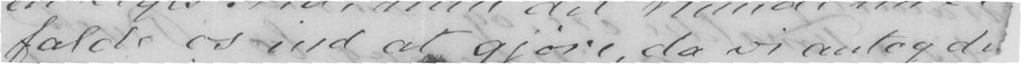falde os ind at gjøre, da vi antog de
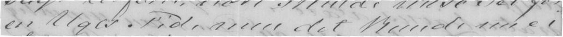en Uges Tid, men det kunde nu ei
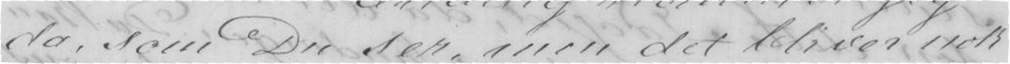da, som Du ser, men det bliver nok
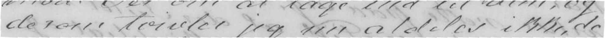derom tvivler jeg nu aldeles ikke, da
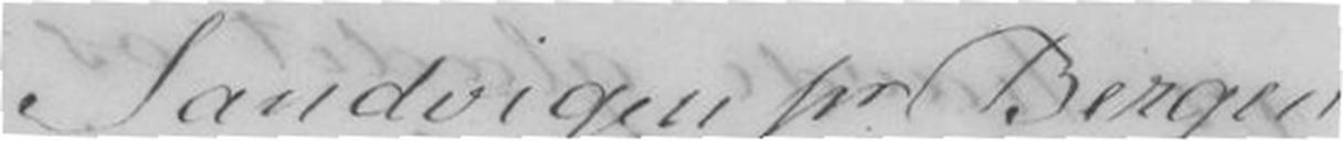Sandvigen pr. Bergen
